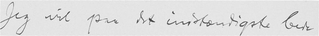Jeg vil paa det indstændigste bede
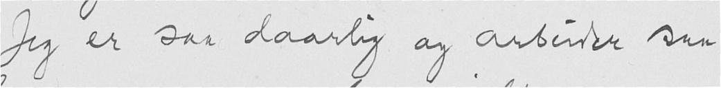Jeg er saa daarlig og arbeider saa
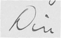Din
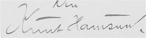Knut Hamsun.
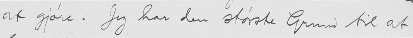at gjøre. Jeg har den største Grund til at
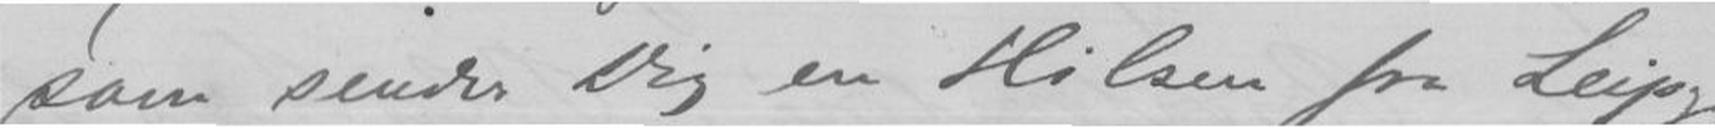sender Dig en Hilsen fra Leipzig.
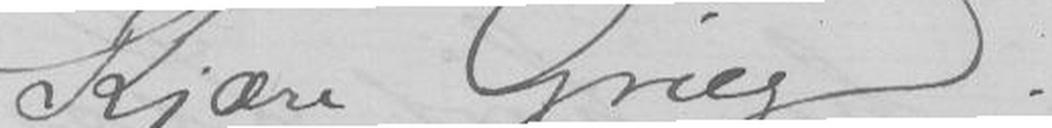Kjære Grieg!
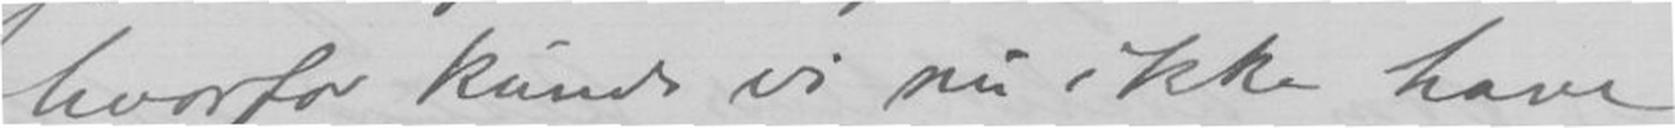hvorfor kunde vi nu ikke have
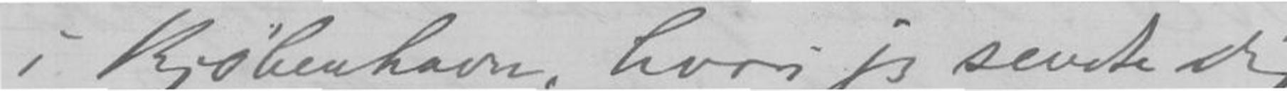i Kjøbenhavn hvori jeg sendte Dig
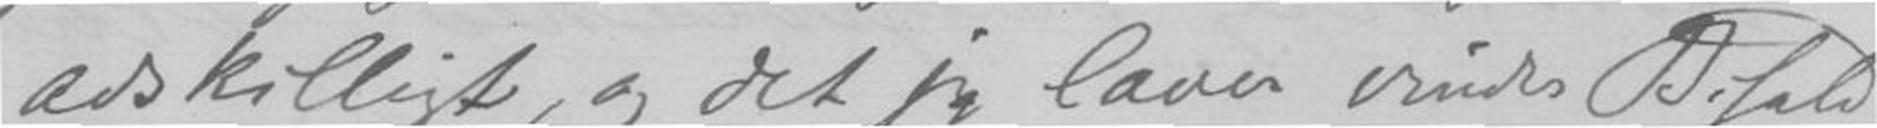adskilligt, og det jeg laver vindes Bifald,
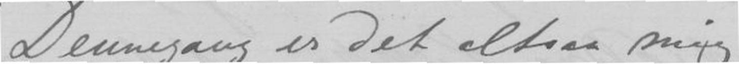Dennegang er Det altsaa mig som
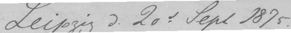Leipzig d. 20d Sept 1875.
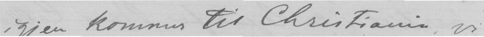igjen kommer til Christiania vi
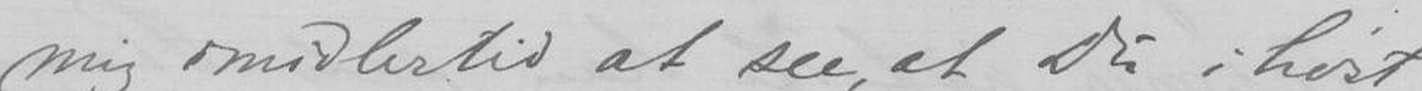mig imidlertid at see, at Du i Høst
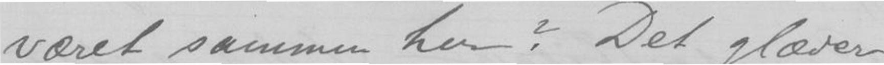været sammen her? Det glæder
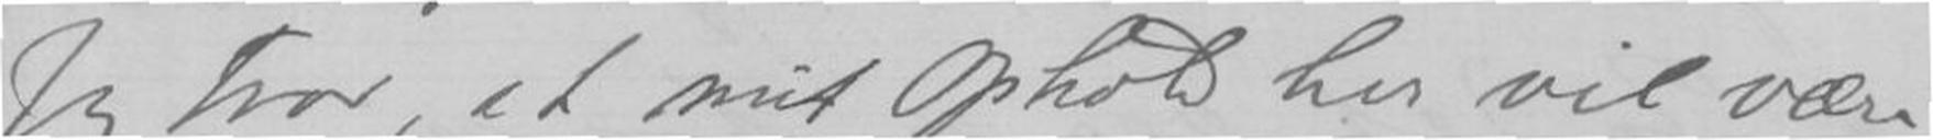Jeg tror, at mit Ophold her vil være
